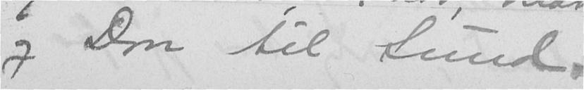og Don til Sund.
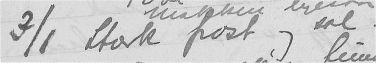3/1 Stærk frost og sol.
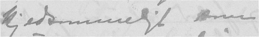kjedsommeligt som
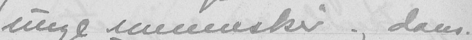unge mennesker og dans.
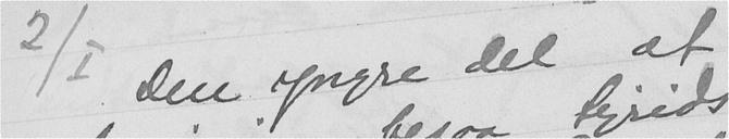2/I Den yngre del af
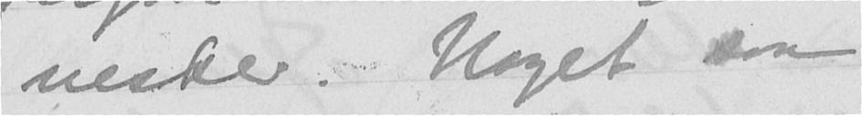nesker. Noget saa
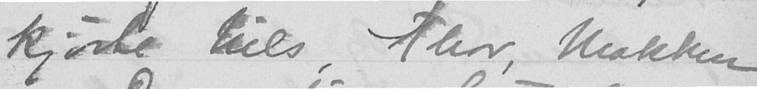kjørte Nils, Thor, Makken
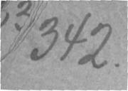342.
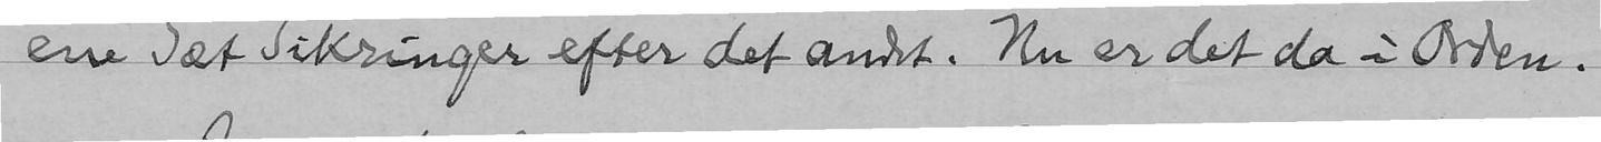ene Sæt Sikringer efter det andet. Nu er det da i Orden.
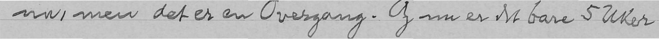nu, men det er en Overgang. Og nu er det bare 5 Uker
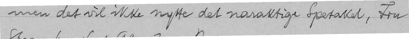men det vil ikke nytte det naraktige Spetakel, Fru
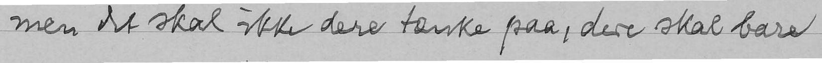men det skal ikke dere tænke paa, dere skal bare
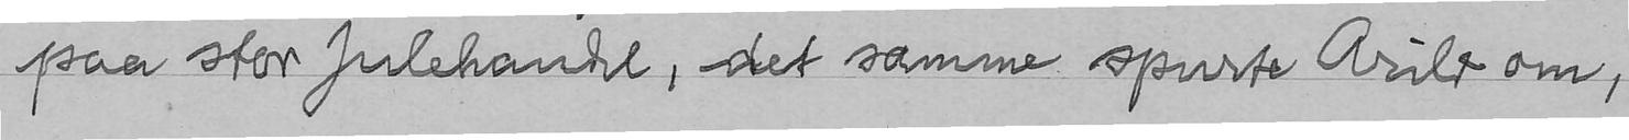paa stor Julehandel, det samme spurte Arild om,
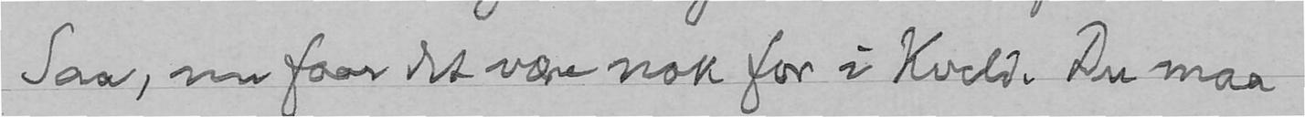Saa, nu faar det være nok for i Kveld. Du maa
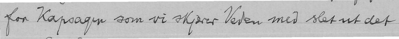for Kapsagen som vi skjærer Veden med slet ut det
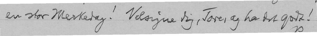en stor Merkedag! Velsigne dig, Tore, og ha det godt!
